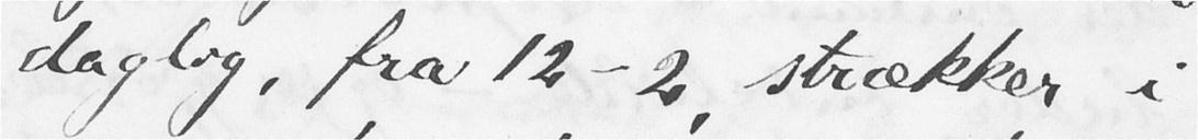daglig, fra 12-2, strækker i
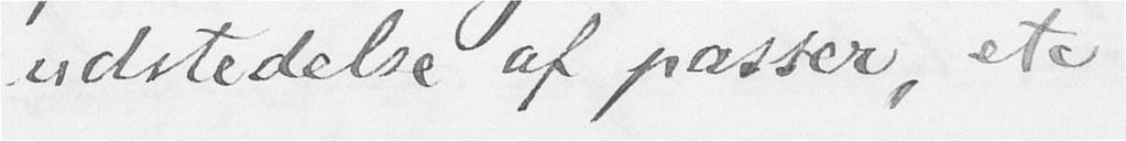udstedelse af passer, etc.
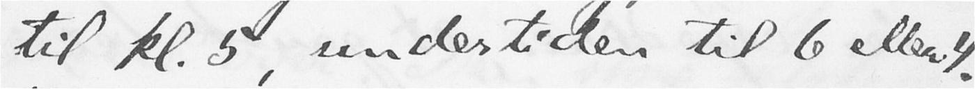til kl. 5, undertiden til 6 eller 4.
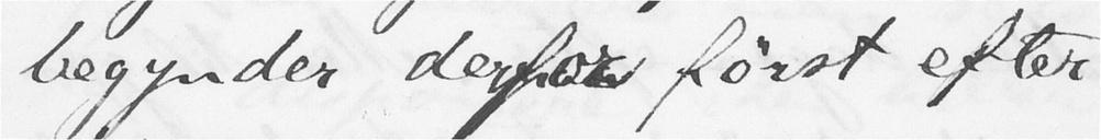begynder derfor først efter
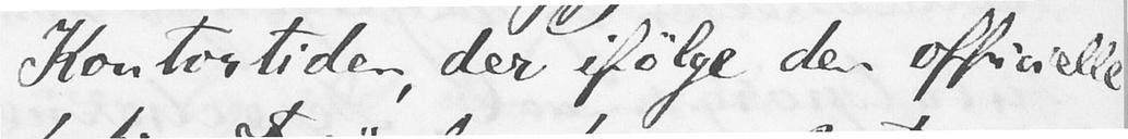Kontortiden, der ifølge den officielle
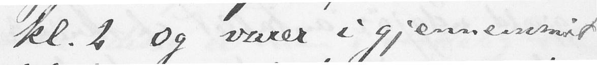kl. 2. og varer i gjennemsnit
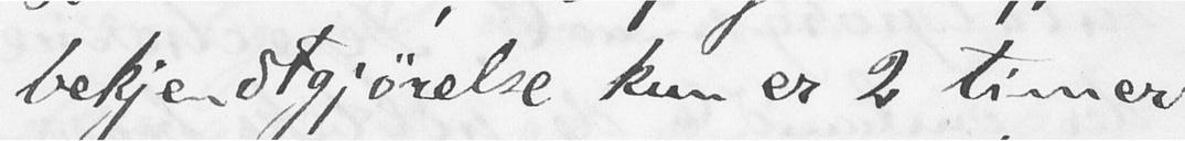bekjendtgjørelse kun er 2 timer
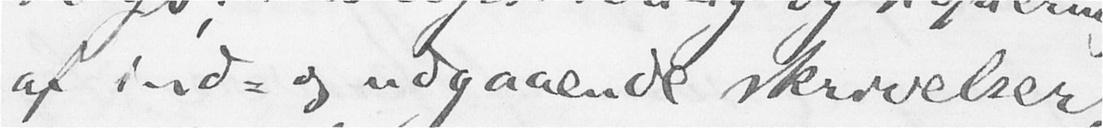af ind- og udgaaende skrivelser,
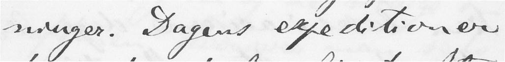ninger. Dagens expeditioner
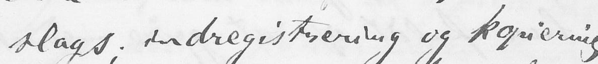slags; indregistrering og kopiering
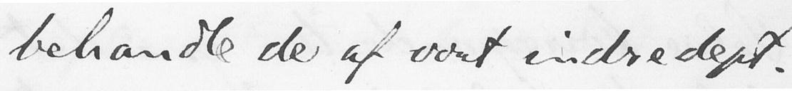behandle de af vort indredept.
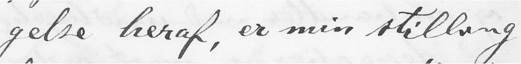gelse heraf, er min stilling
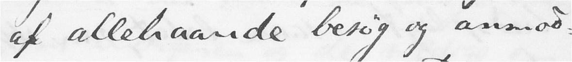af allehaande besøg og anmod-
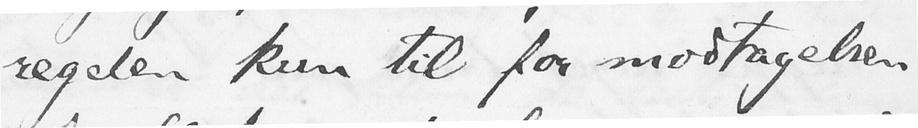regelen kun til for modtagelsen
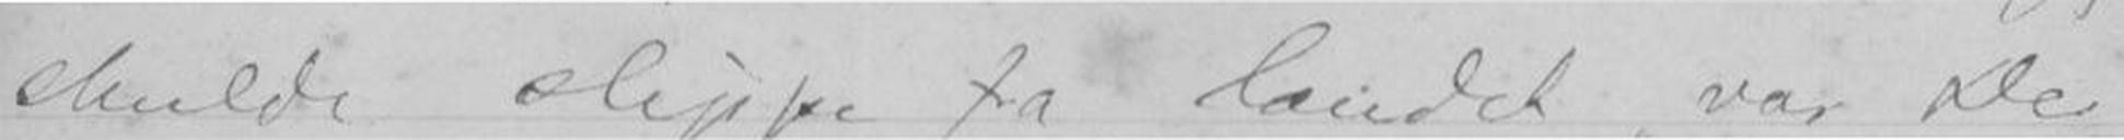skulde slippe fra landet, var De
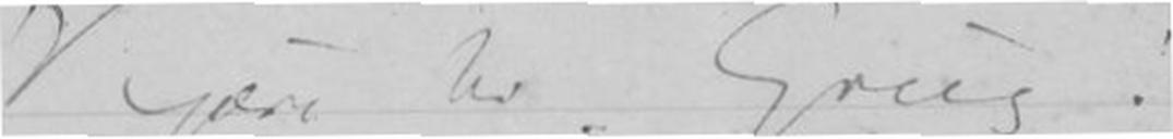Kjære hr. Grieg!
